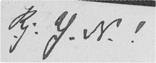Kj. Y. N.!
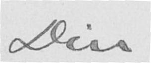Din
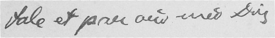tale et par ord med Dig.
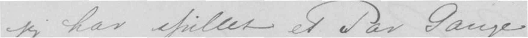jeg har spillet et Par Gange
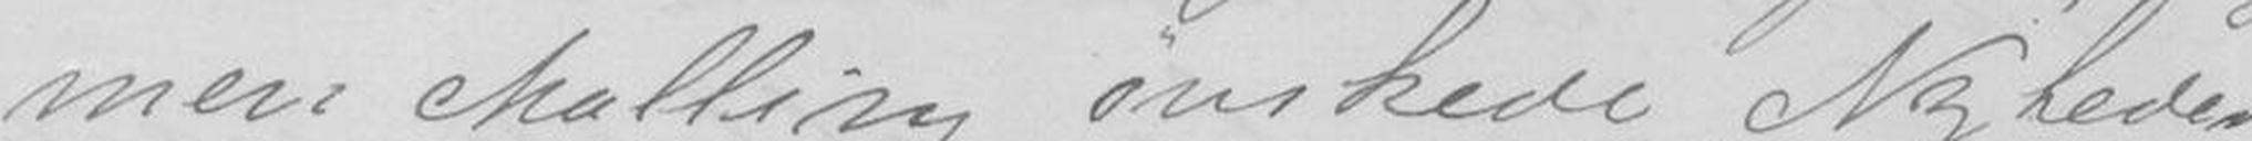men ønskede "Nyheder".
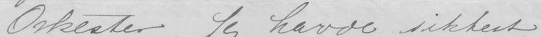Orkester. Jeg havde sikkert
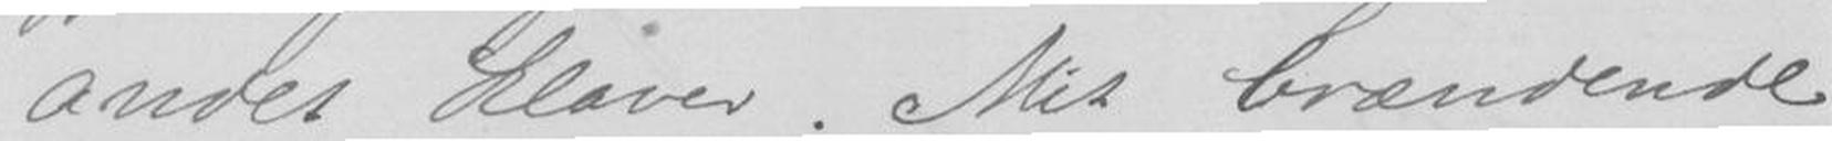andet klaver. Mit brændende
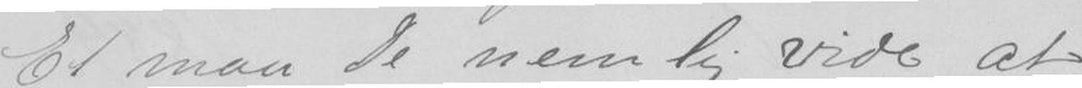Et maa De nemlig vide, at
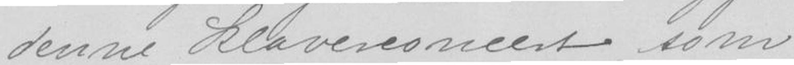denne Klaverconcert, som
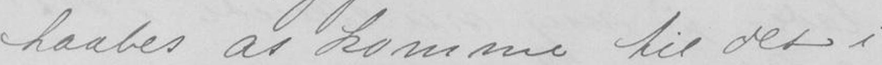haabet at komme til det i
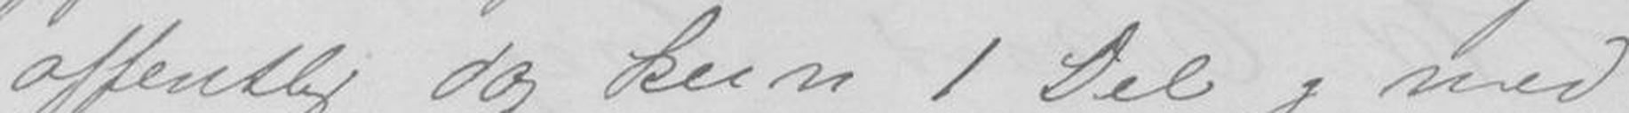offentlig dog kun 1 Del og med
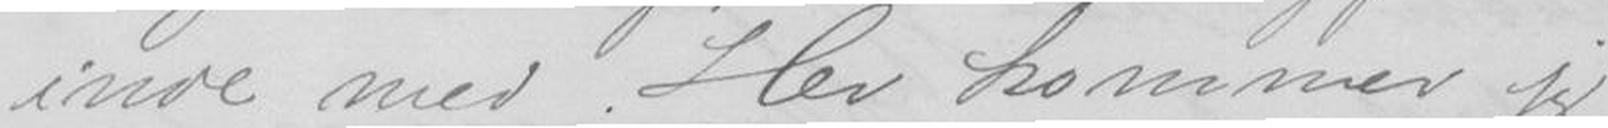inde med. Her kommer jeg
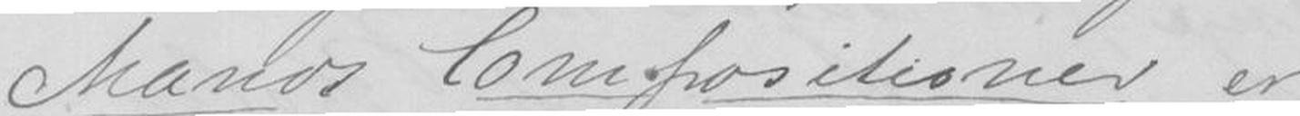Mands Compositioner er
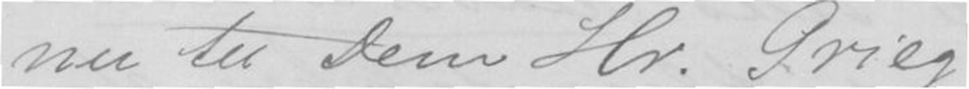nu til Dem Hr. Grieg!,
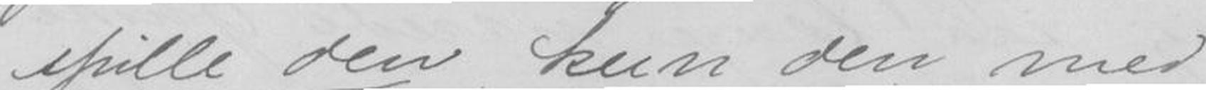spille den, kun den med
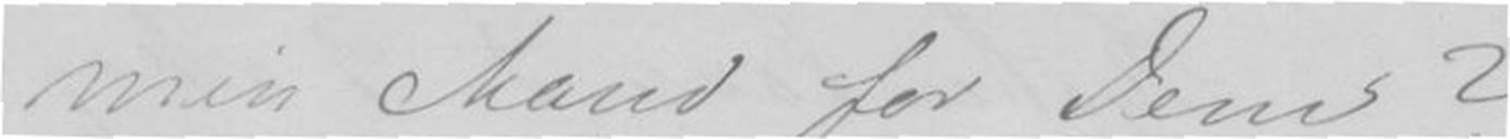min Mand for Dem?
